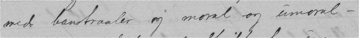med banstraaler og moral og umoral —
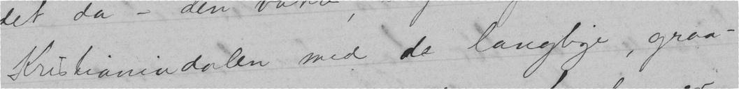Kristianiadalen med de langlige, graa-
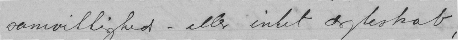samvittigheds- eller intet ægteskab,
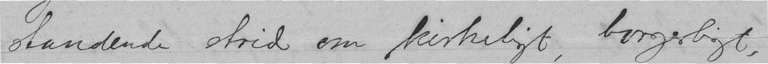standende strid om kirkeligt, borgerligt,
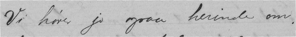Vi hører jo ogsaa herinde om,
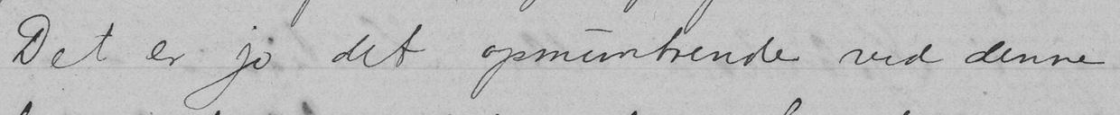Det er jo det opmuntrende ved denne
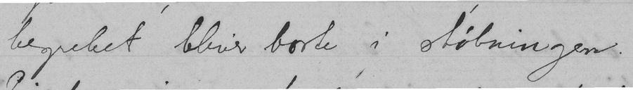begrebet bliver borte i støbningen.
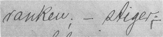ranken; - stiger;
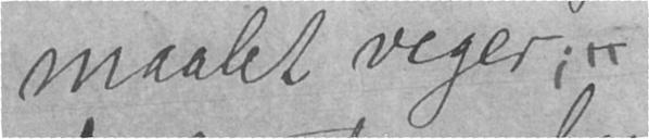maalet viger; -
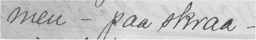men - paa skraa -
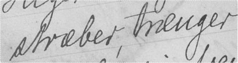stræber, trenger
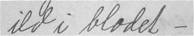ild i blodet -
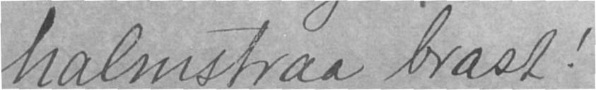halmstraa brast!
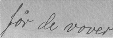før de vover
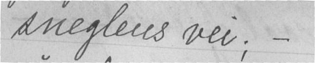sneglens vei; -
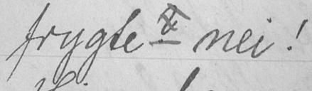frygte? - nei!
